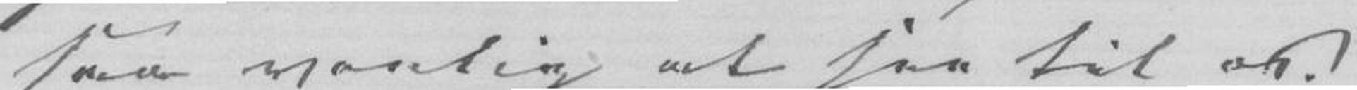saa vænlig at see til os.
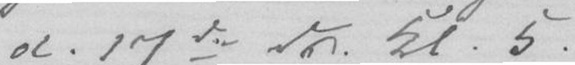d. 17de Ds. kl. 5.
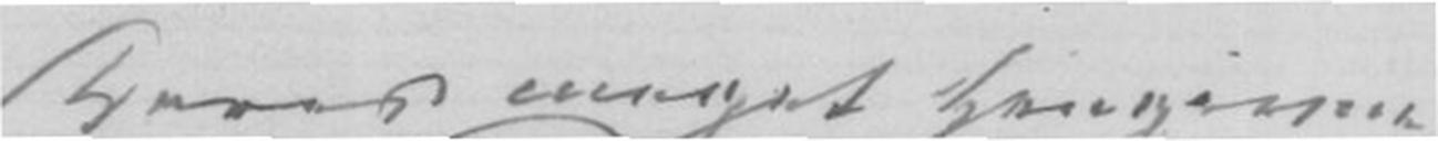Deres meget hengivne
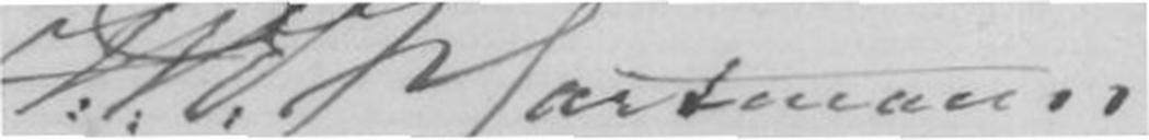J. P. E. Hartmann
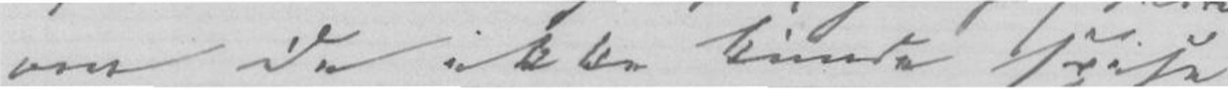om De ikke kunde spise
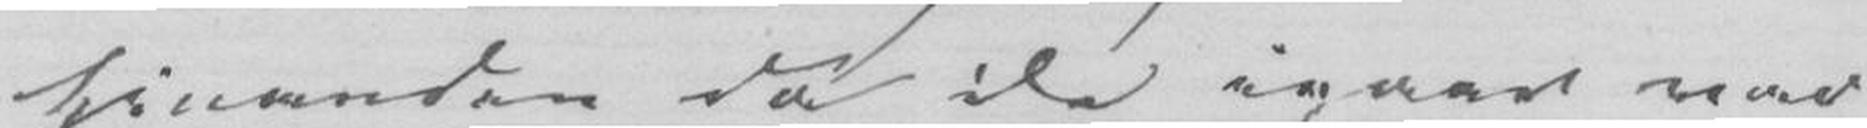hinanden da De igaar var
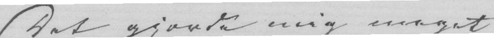Det gjorde mig meget
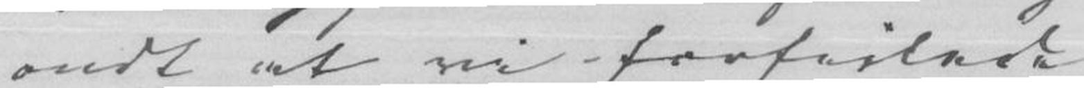ondt at vi forfeilede
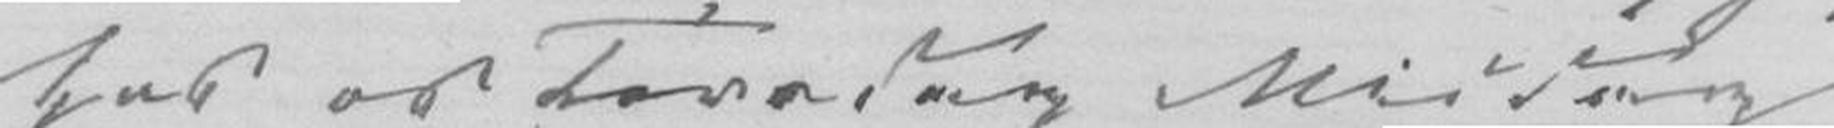hos Torsdag Middag
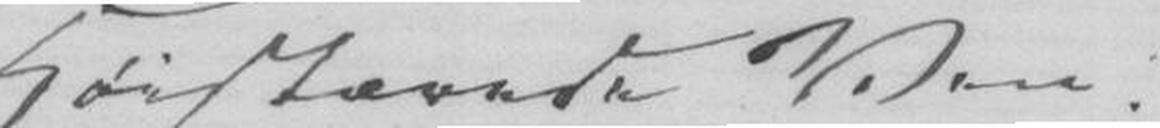Høistærede Ven!
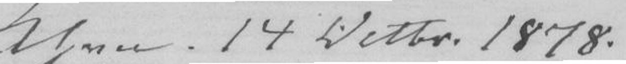Khvn. 14. Octbr. 1878
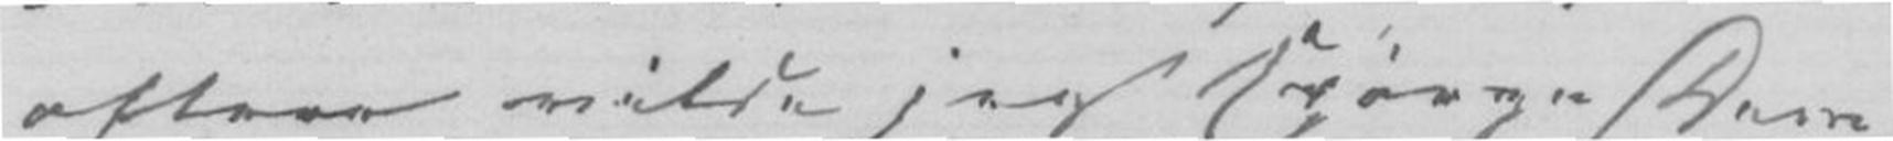oftere vilde jeg spørge Dem
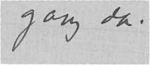gang da.
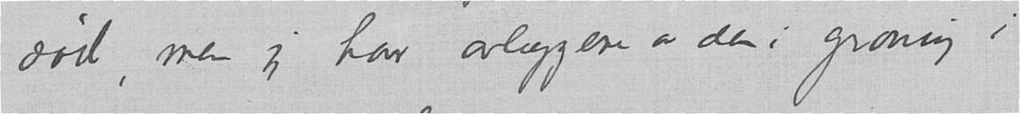død, men jeg har avlæggere av den i groning i
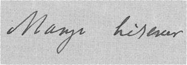Mange hilsener
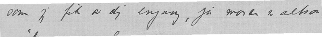som jeg fik av dig engang, ja moren er altsaa
